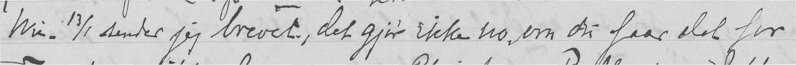Nu, 13/1 sender jeg brevet, det gjør ikke no, om du faar det for
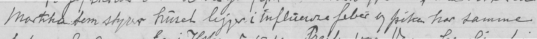Martha som styrer huset ligger i influenzafeber og piken har samme
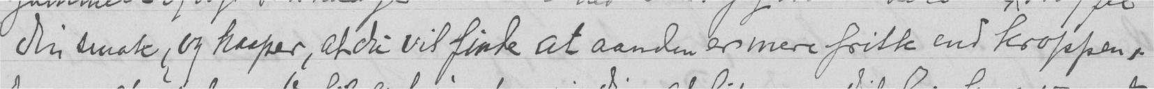din smak, og haaper, at du vil finde at aanden er mere frisk end kroppen,
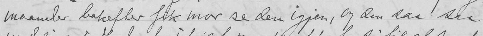maaneder bakefter fik mor se den igjen, og den saa saa
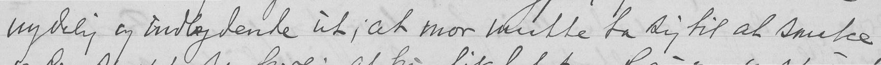nydelig og indbydende ut, at mor maatte ta sig til at smake
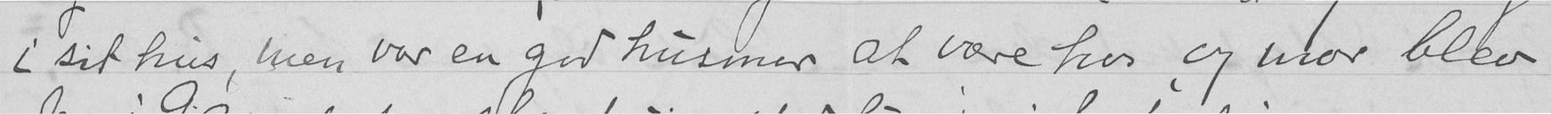i sit hus, men var en god husmor at være hos, og mor blev
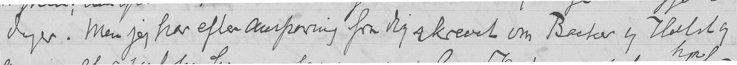dager. Men jeg har efter ansporing fra dig skrevet om Backer og Holst og
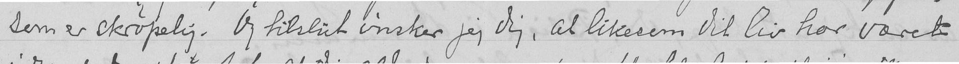som er skrøpelig. Og tilslut ønsker jeg dig, at likesom dit liv har været
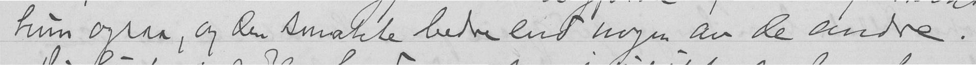hun ogsaa, og den smakte bedre end nogen av de andre.
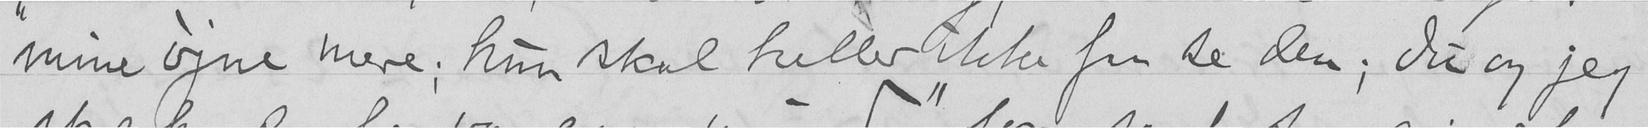mine øine mere; hun skal heller ikke faa se den; du og jeg
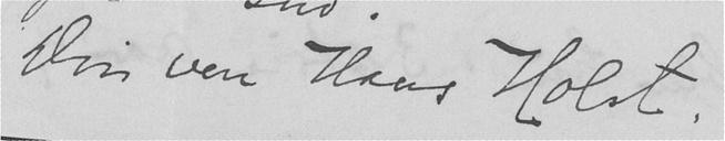Din ven Hans Holst.
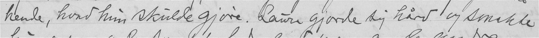hende, hvad hun skulde gjøre. Laura gjorde sig hård og smakte
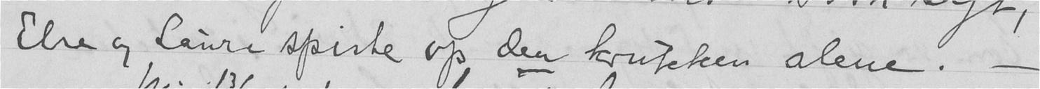Else og Laura spiste op den krukken alene. -
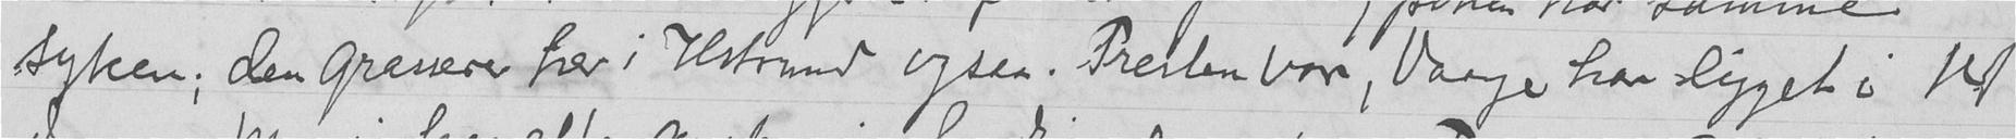syken; den grasserer her i Hstrand ogsaa. Presten vor, Vaage har ligget i 14
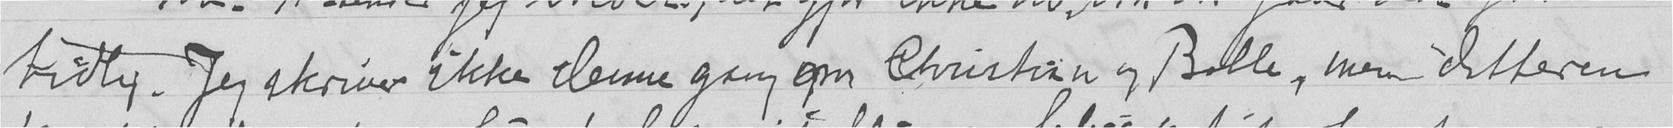tidlig. Jeg skriver ikke denne gang om Christian og Bolle, men datteren
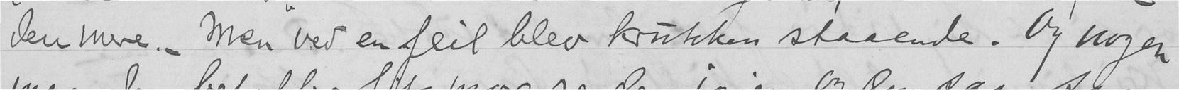den mere. - Men ved en feil blev krukken staaende. Og nogen
